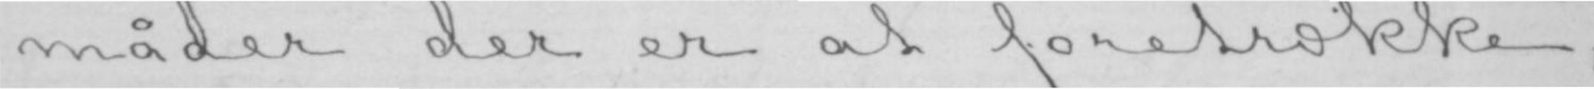måder der er at foretrække,
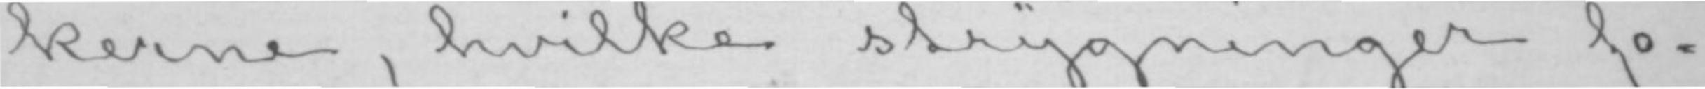kerne, hvilke strygninger fo-
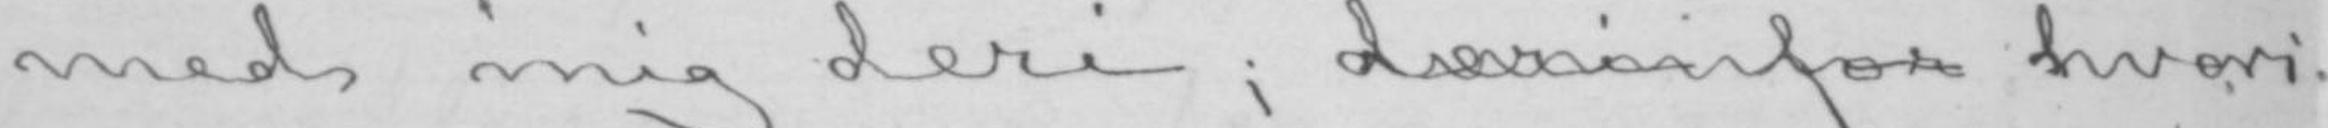med mig deri; deri for hvori-
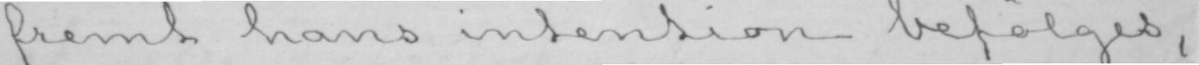fremt hans intention befølges,
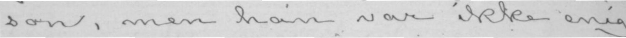son, men han var ikke enig
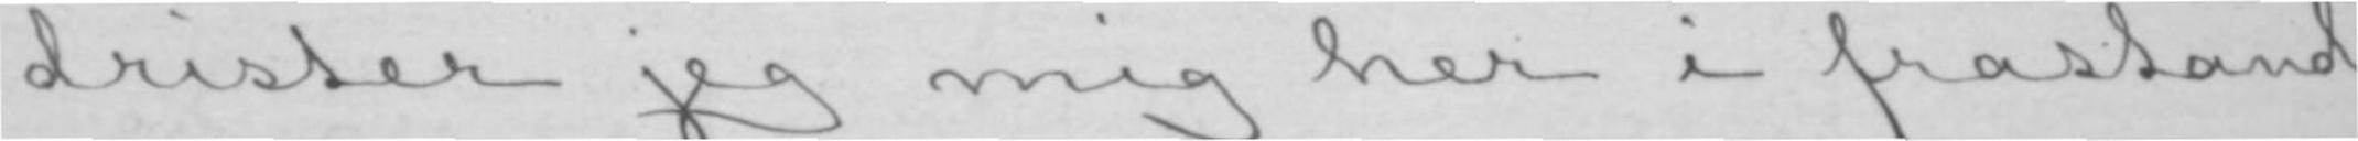drister jeg mig her i frastand
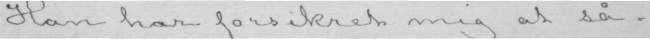Han har forsikret mig at så-
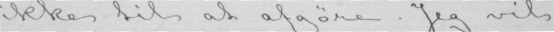ikke til at afgøre. Jeg vil
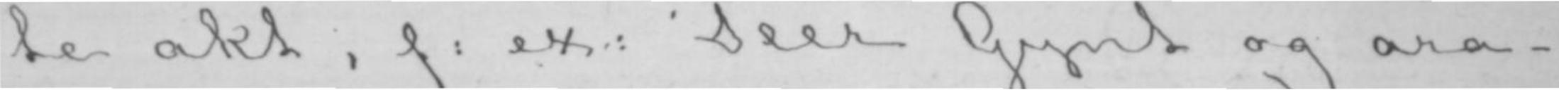te akt, f: ex: Peer Gynt og ara-
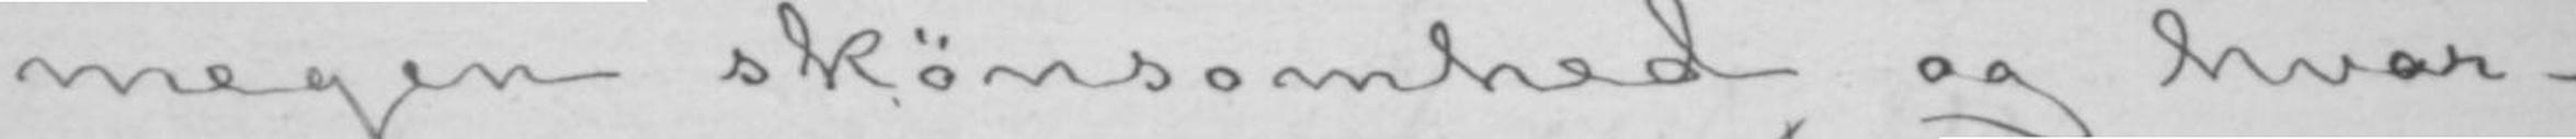megen skønsomhed og hvor-
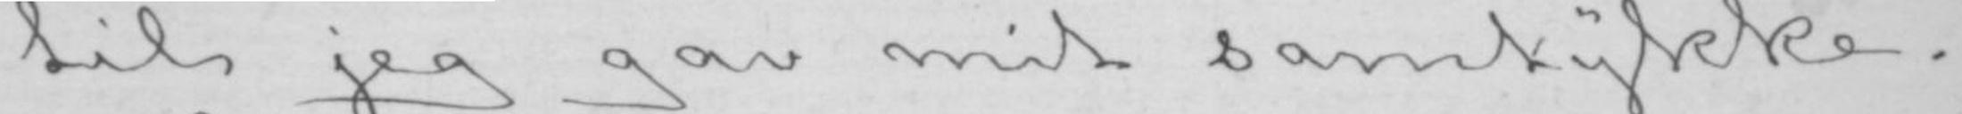til jeg gav mit samtykke.
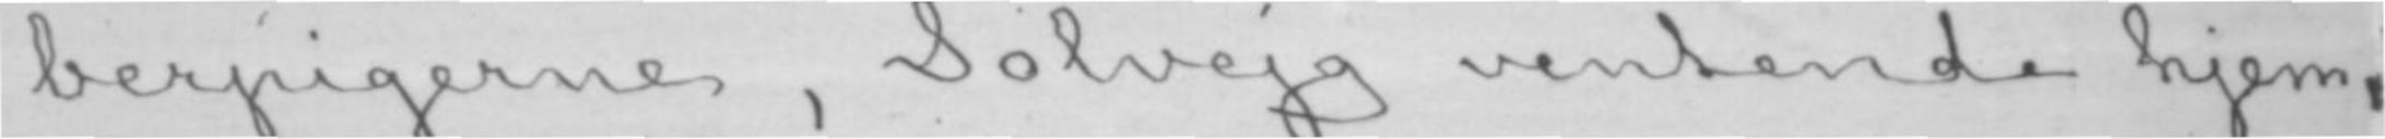berpigerne, Solvejg ventende hjem-
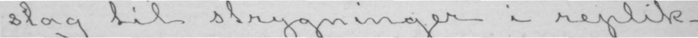slag til strygninger i replik-
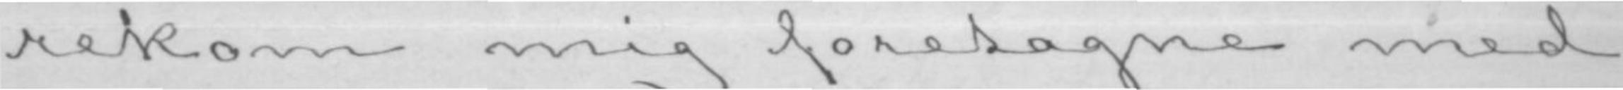rekom mig foretagne med
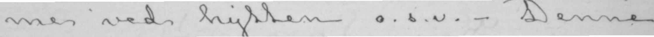me ved hytten o. s. v.- Denne
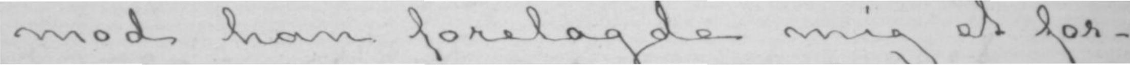mod han forelagde mig et for-
